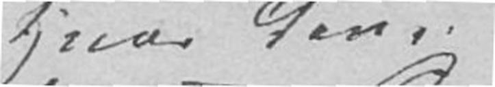Hvor denne
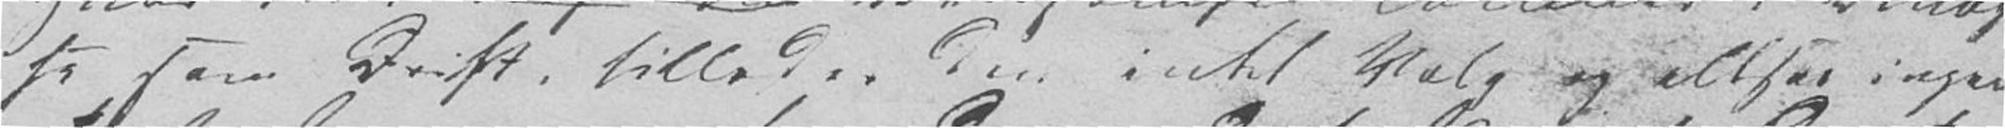se som Drift, tillader den intet Valg og altsaa ingen
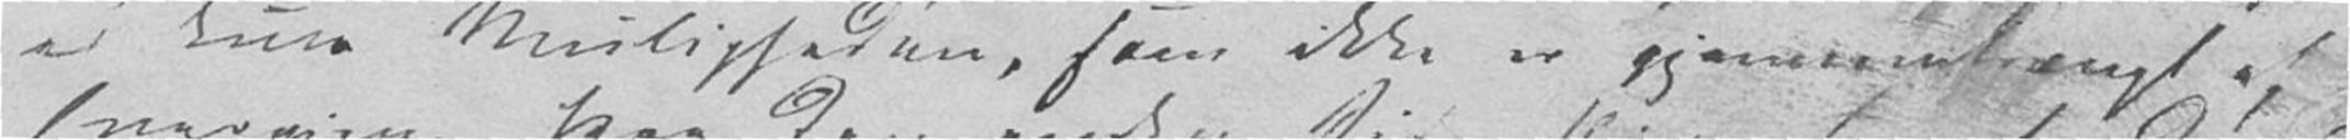er kun Muligheden, som ikke er gjennemtrængt af
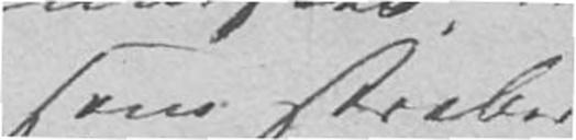som stræber
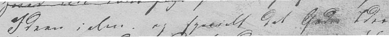Ideen i alm. og specielt det Godes Idee
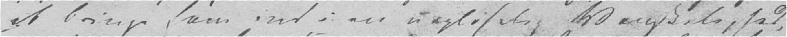at bringe ham ind i en uopløselig Vanskelighed,
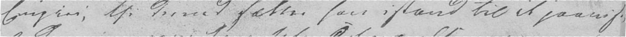Empiri, thi derved sættes han istand til at paavise,
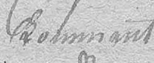komment
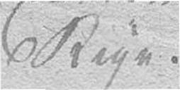Rige.
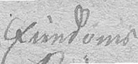Eiendoms
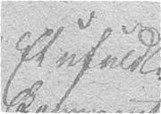Et ufuld-
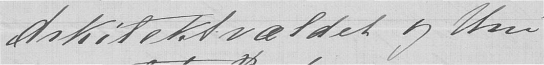Arkitektvældet og Uni-
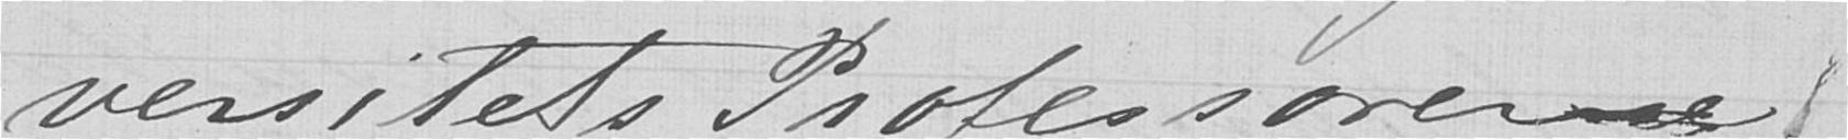versitets Professorene!
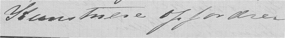Kunstnere opfordrer
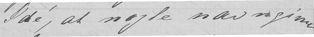Idé, at nogle navngivne
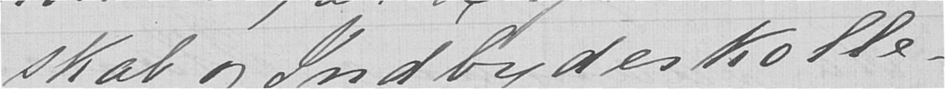skab og Indbydeskolle-
